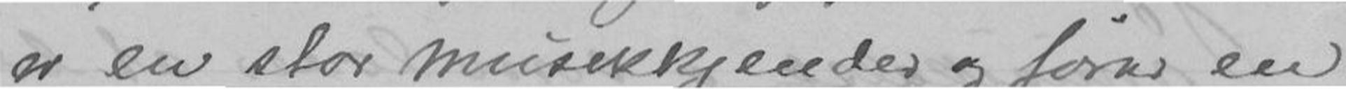er en stor musikkjender og fører en
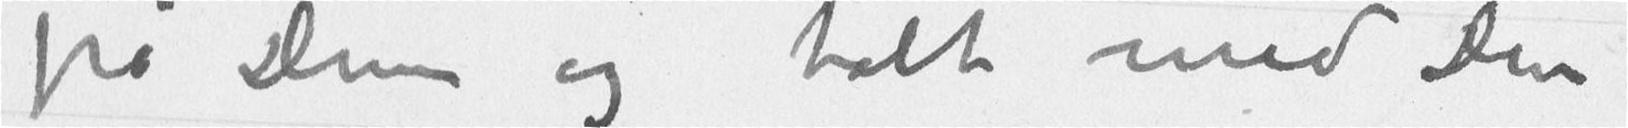på Dem og talt med Dem
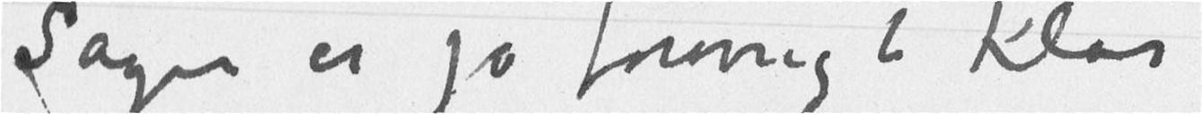Sagen er jo formeligt klar
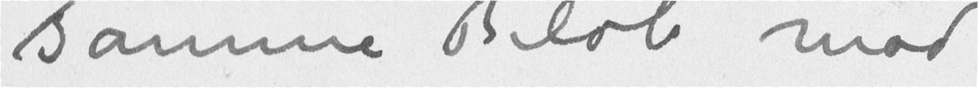samme Beløb mod
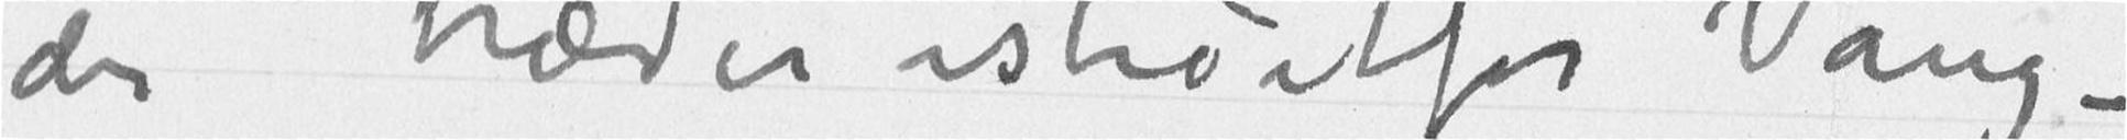der træder istedetfor Vang –
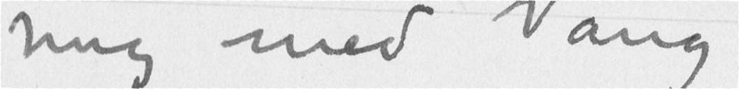mig med Vang
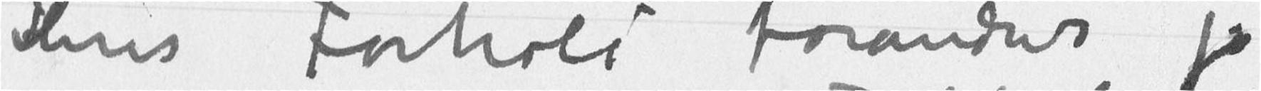Deres Forhold forandres jo
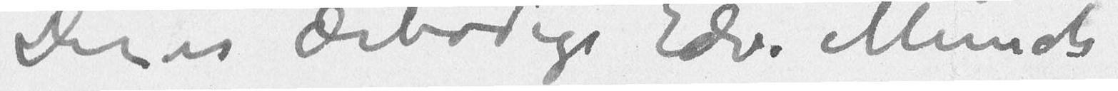Deres ærbødige Edv. Munch
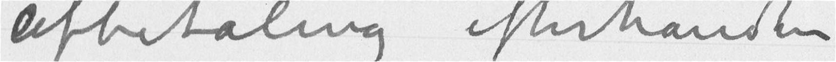afbetaling efterhånden
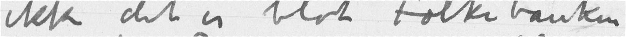ikke det er blot Folkebanken
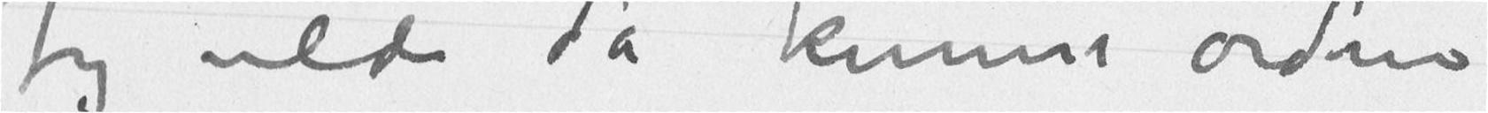Jeg vilde da kunne ordne
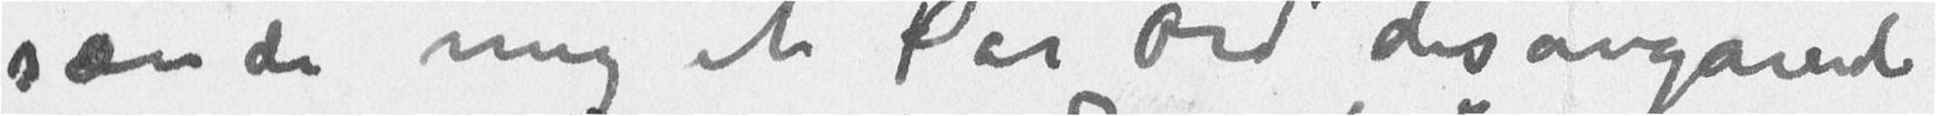sænde mig et Par Ord desangående
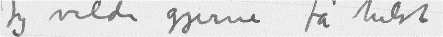Jeg vilde gjerne få hilst
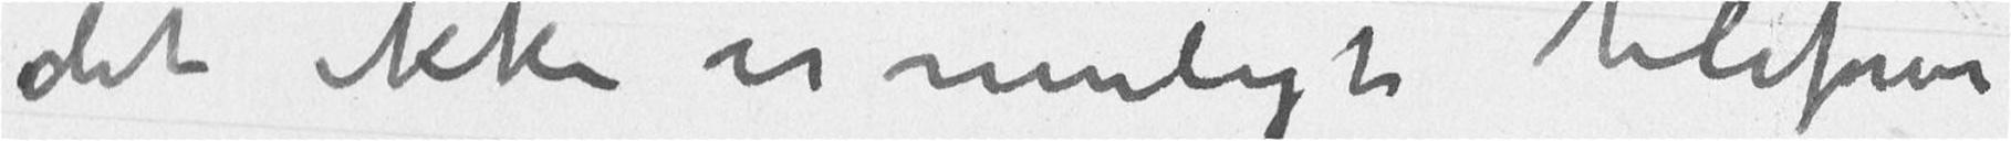det ikke er muligt telefonerer
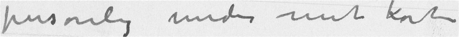personlig under mit korte
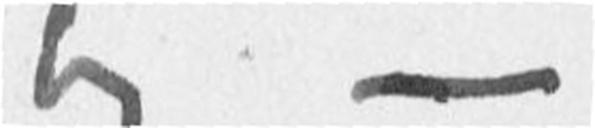jeg –
